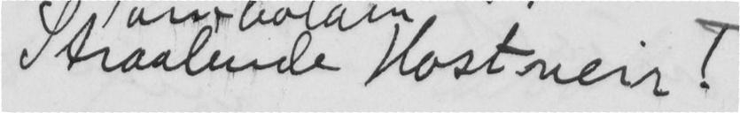Straalende Hostveir!
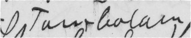Tombolaen
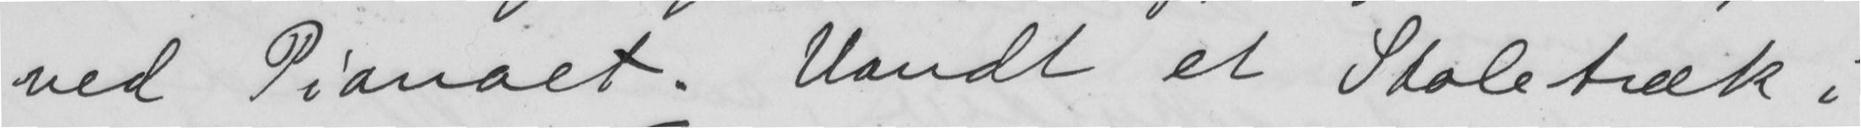ved Pianoet. Vandt et Stoletræk i
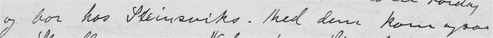og bor hos Steinsviks. Med dem kom ogsaa
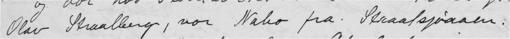Olav Straalberg, vor Nabo fra Straalsjøaaen.
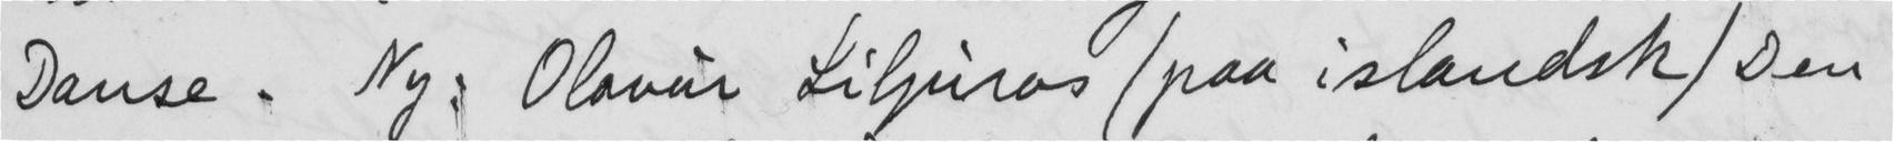Danse. Ny: Olavur Liljuros (paa islandsk) Den
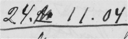24. 11.04
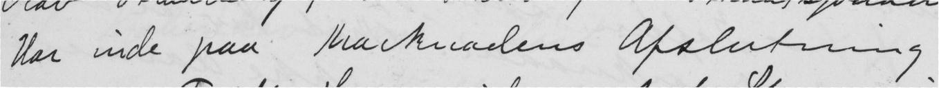Var inde paa Marknadens Afslutning
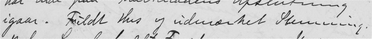igaar. Fuldt Hus og udmærket Stemning.
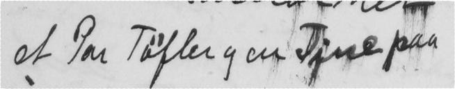et Par Tufler og en Tine paa
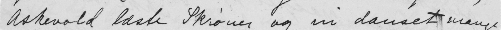Askevold læste Skrøner og vi danset mange
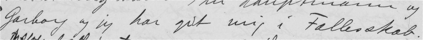Garborg og jeg har git mig i Fællesskab.
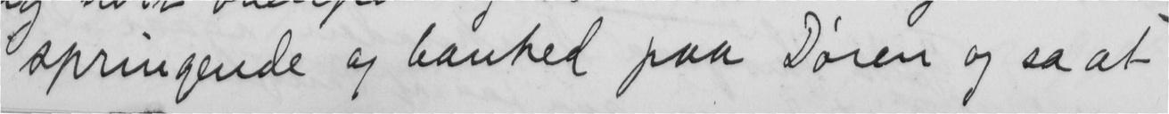springende og banked paa Døren og sa at
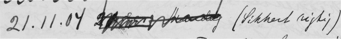21.11.04  (Sikkert rigtig)
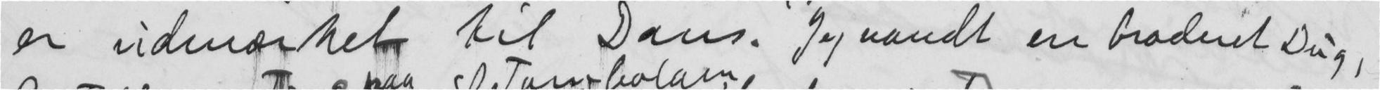er udmærket til Dans. Jeg vandt en broderet Dug,
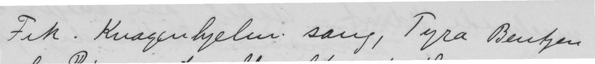Frk. Knagenhjelm sang, Tyra Bentzen
